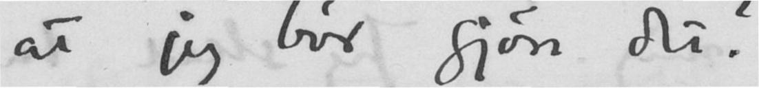at jeg bør gjøre det?
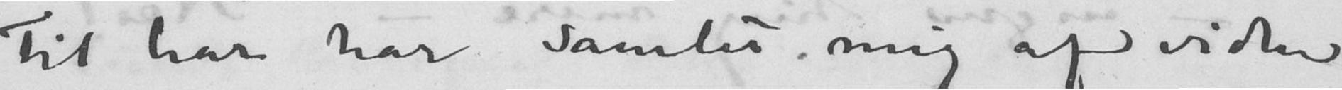til har har samlet mig af viden
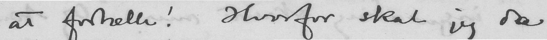at fortælle! Hvorfor skal jeg da
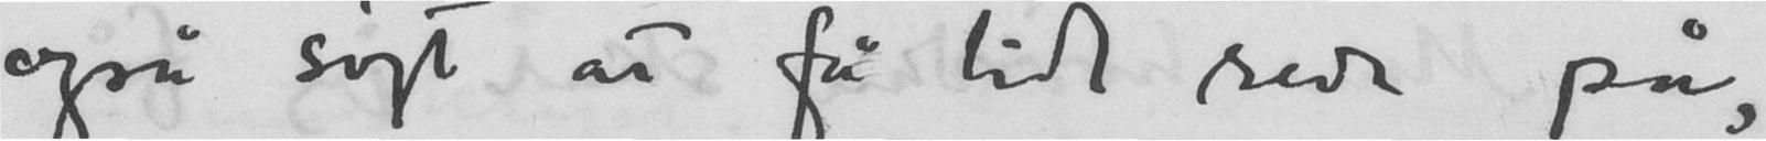også søgt at få lidt rede på,
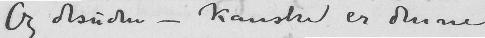Og desuden - kanske er denne
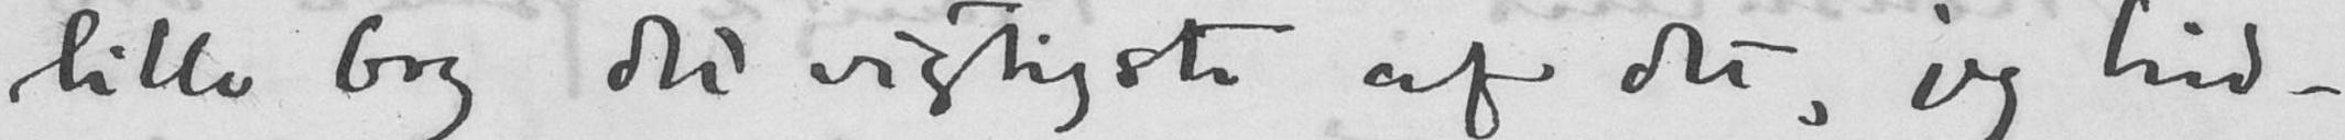lille bog det vigtigste af det, jeg hid-
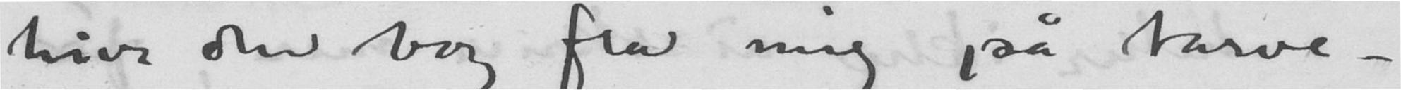hive den bog fra mig på tarve-
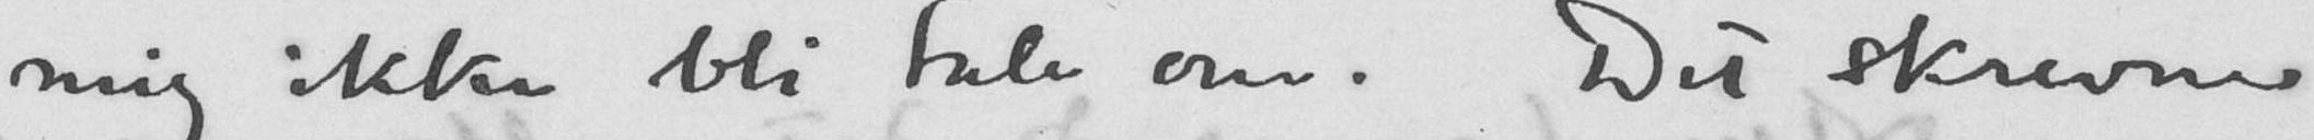mig ikke bli tale om. Det skrevne
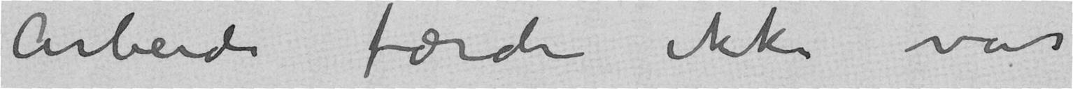Arbeide færdi ikke var
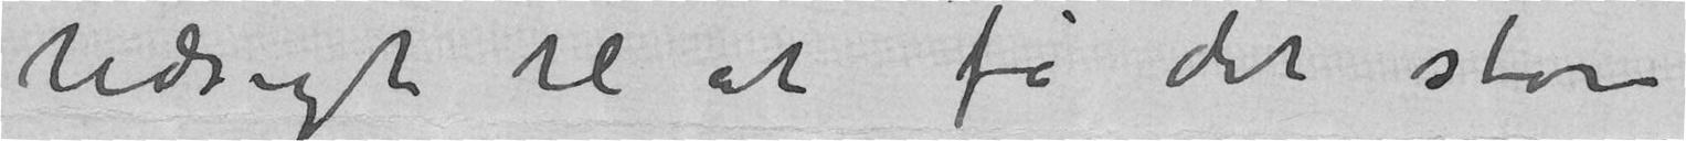Udsigt til at få det store
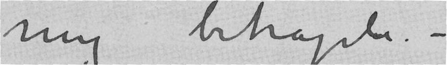mig behageli –
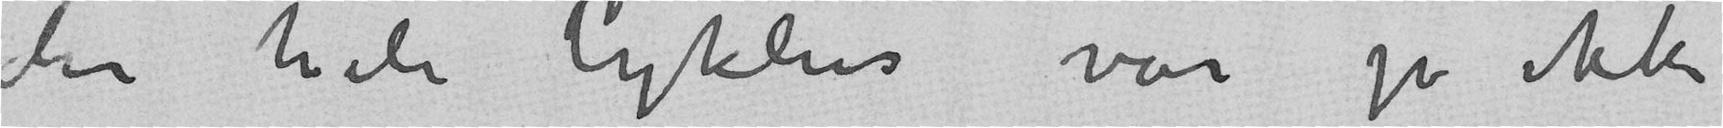den hele Cyklus var jo ikke
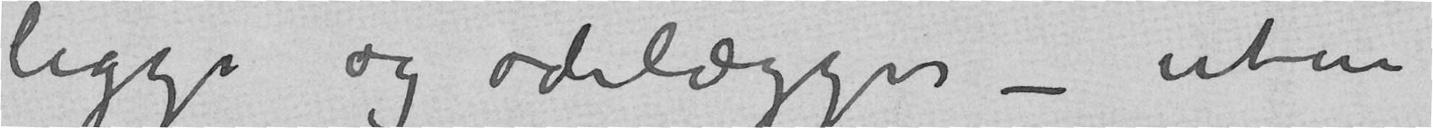ligge og ødelægges – uten
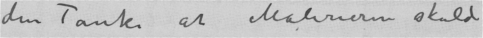den Tanke at Malerierne skulde
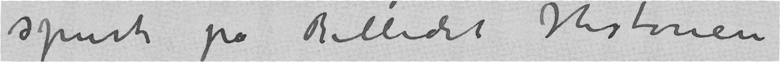spurt på Billedet Historien
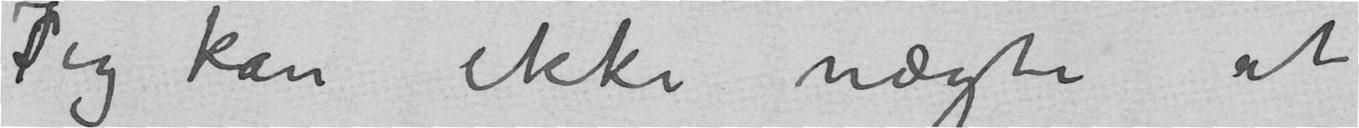Jeg kan ikke nægte at
